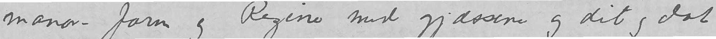maner-form og Regine med gjæssene og dit og dat
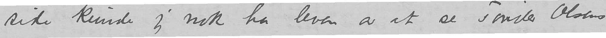side kunde jeg nok ha leven av at se Tønder Olsens
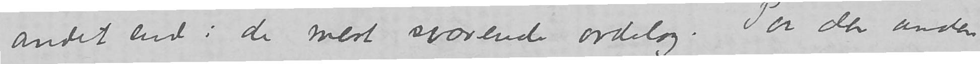andet end i de mest svævende ordelag. Paa den anden
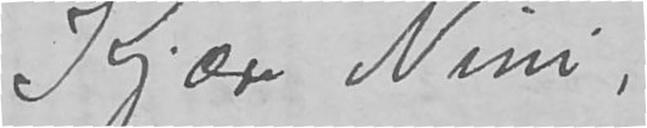Kjære Nini
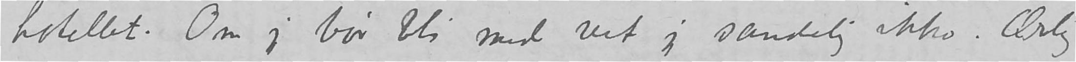hotellet. Om jeg bør bli med vet jeg sandelig ikke. Ærlig
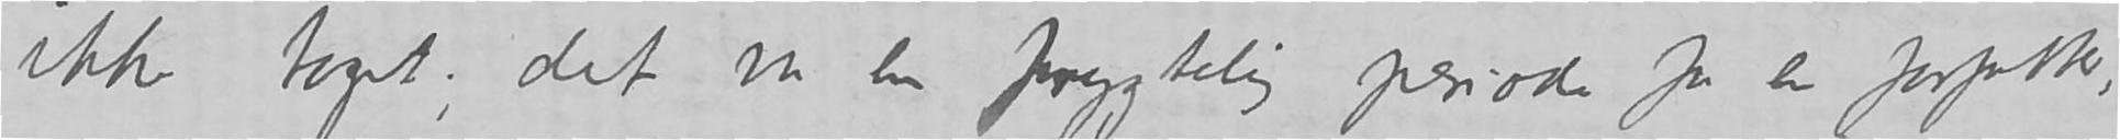ikke toget; det var en frygtelig periode for en forfatter,
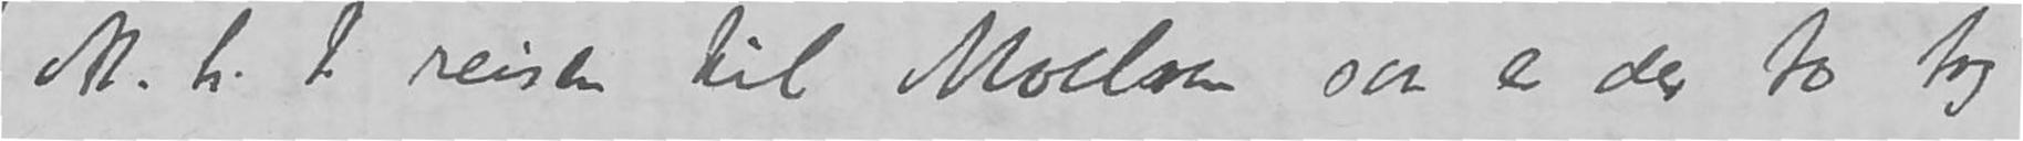M.h.t. reisen til Moelven saa er der to tog
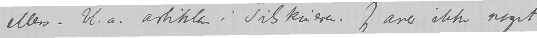ellers . –  bl.a. artiklen i Tilskueren. Jeg aner ikke noget
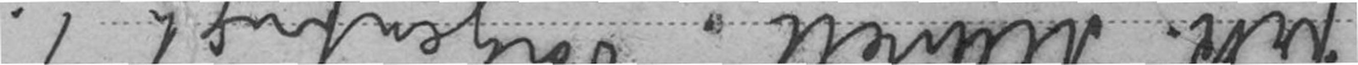frk. Munch. Sorgenfrigt 1.
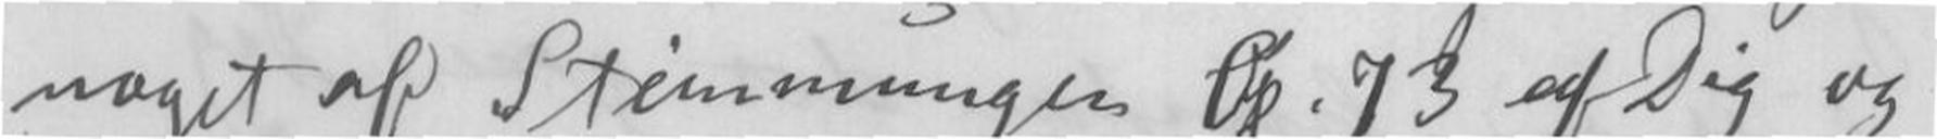noget af Stimmungen Op. 73 af Dig og
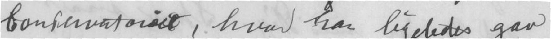Concervatoriet, hvor hun ligeledes gav
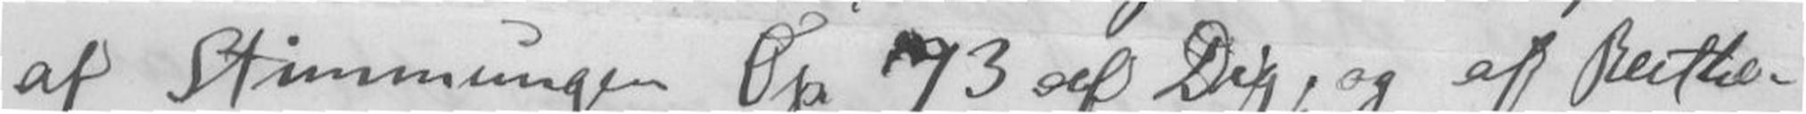af Stimmungen Op. 73 af Dig, og af Beetho-
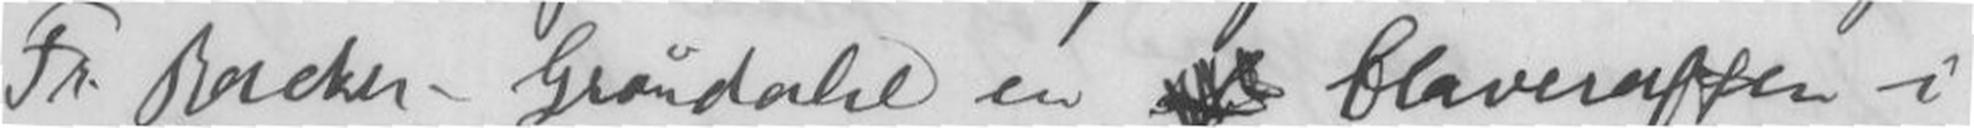Fr. Backer-Grøndahl en Claveraften i
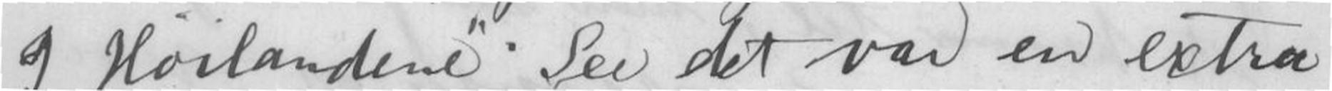I Høilandene. See det var en extra
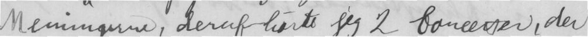Meningerne, deraf hørte jeg 2 Concerter, der
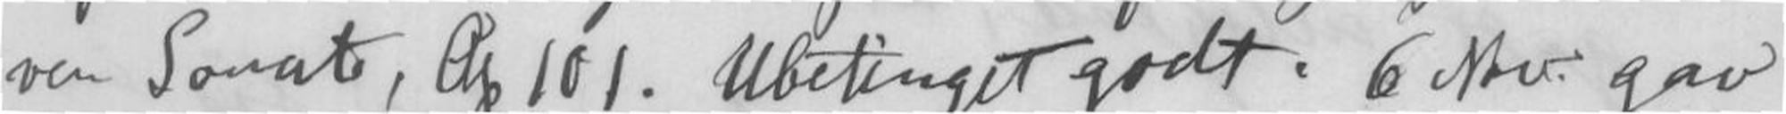ven Sonate, Op 101. Ubetinget godt. 6 Nov. gav
